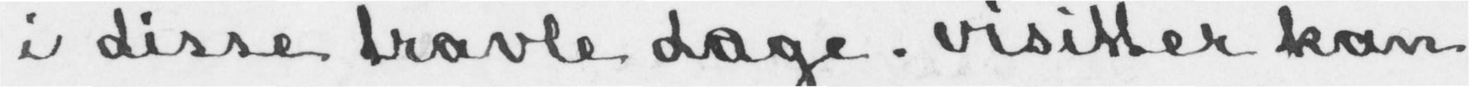i disse travle dage. visitter kan
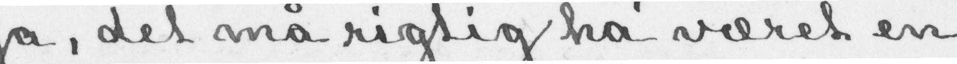Ja, det må rigtig ha' været en
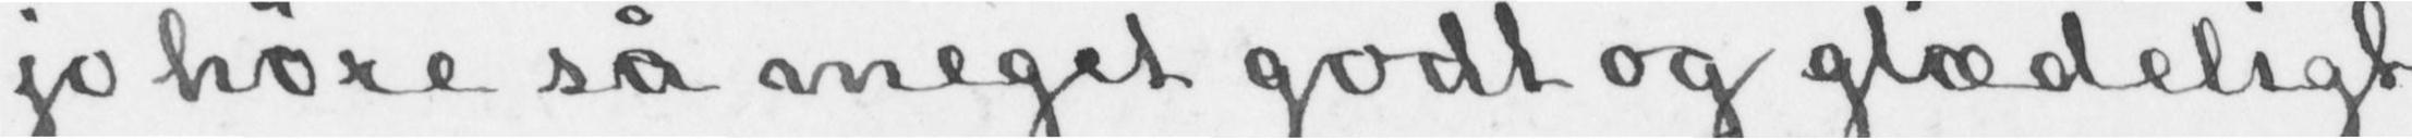jo høre så meget godt og glædeligt
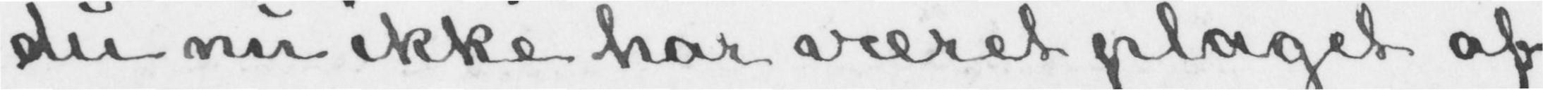du nu ikke har været plaget af
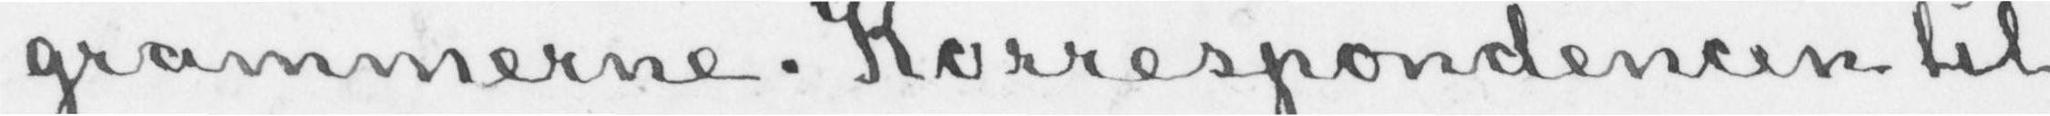grammerne. Korrespondencen til
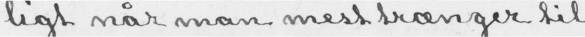ligt når man mest trænger til
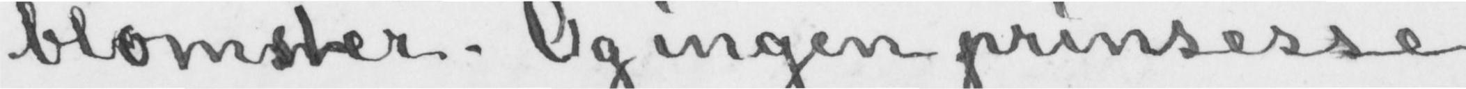blomster. Og ingen prinsesse
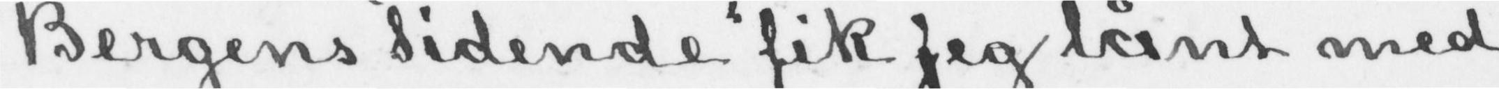«Bergens Tidende» fik jeg lånt med
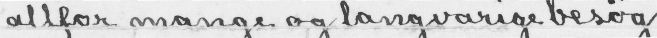altfor mange og langvarige besøg
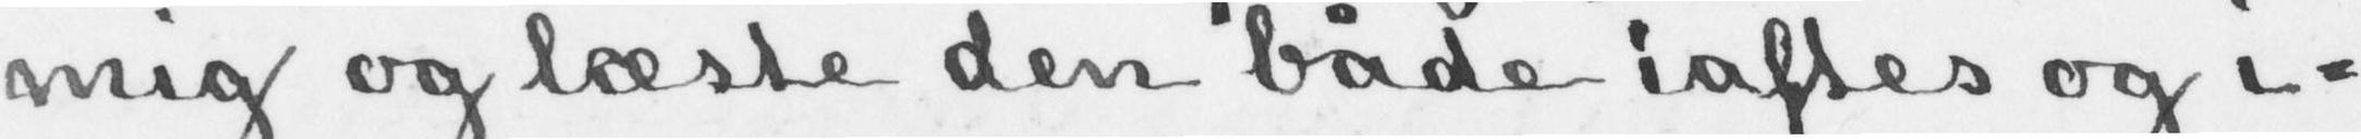mig og læste den både iaftes og i-
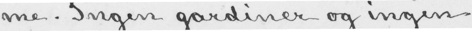me. Ingen gardiner og ingen
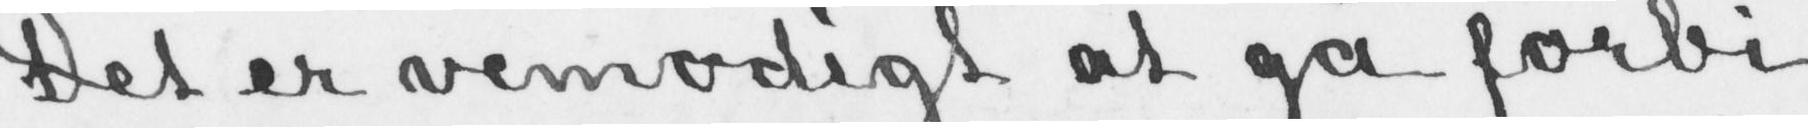Det er vemodigt at gå forbi
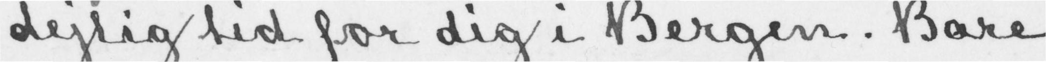dejlig tid for dig i Bergen. Bare
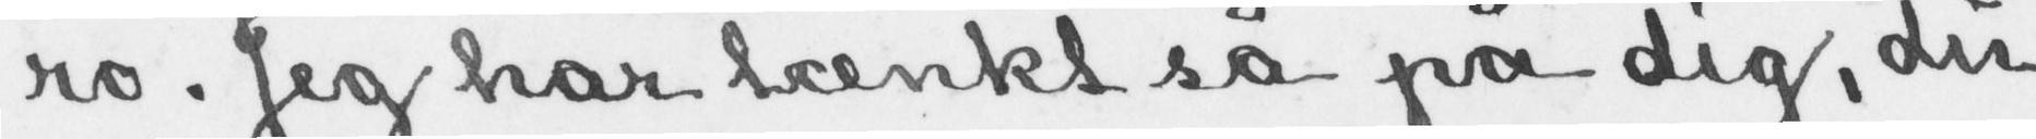ro. Jeg har tænkt så på dig, du
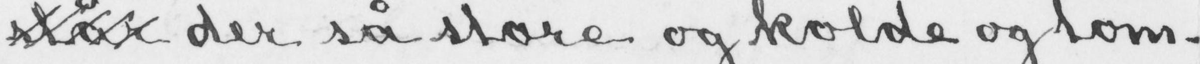står der så store og kolde og tom-
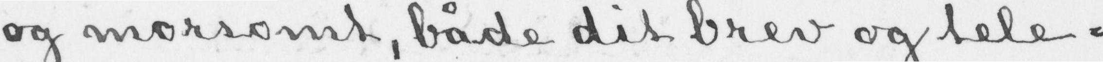og morsomt, både dit brev og tele-
